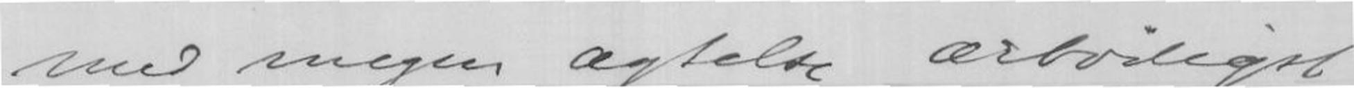Med megen agtelse ærbødigst
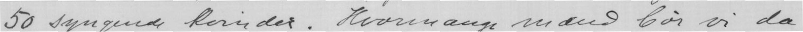50 syngende kvinder. Hvor mange mænd bør vi da
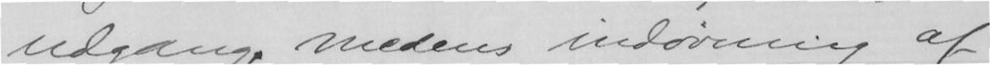udgang, medens indøvning af
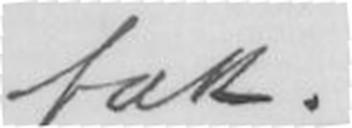tak.
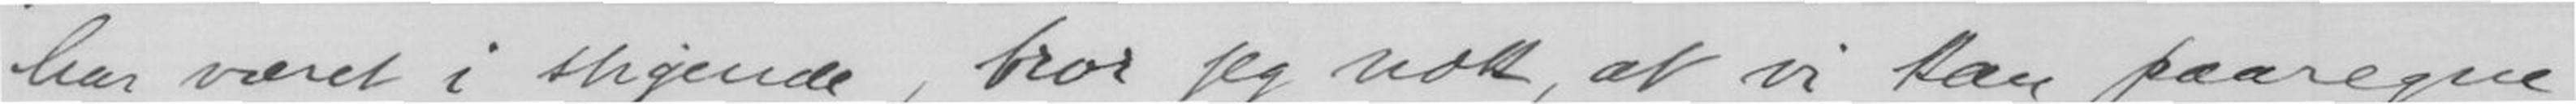har været i stigende, tror jeg nok, at vi kan paaregne
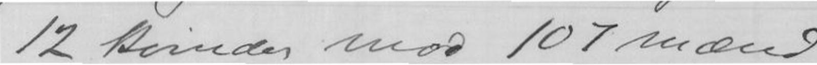12 Kvinder mod 107 mænd,
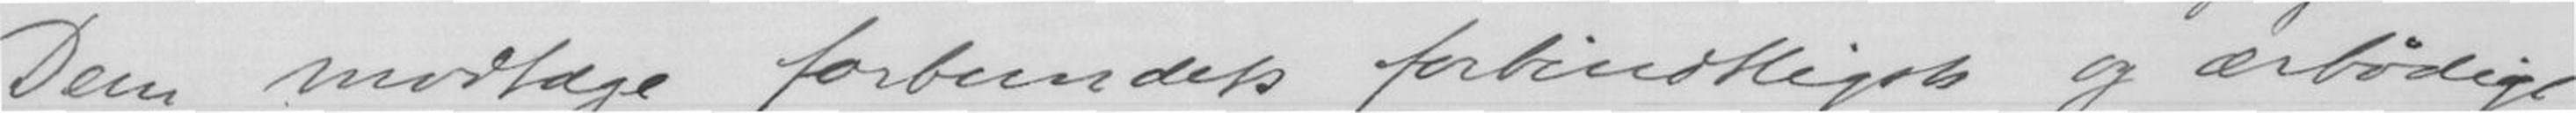Dem modtage forbundets forbindtligste og ærbødige
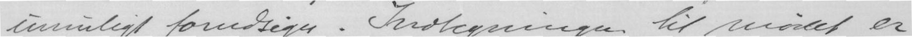umuligt forudsiges. Indtegningen til mødet er
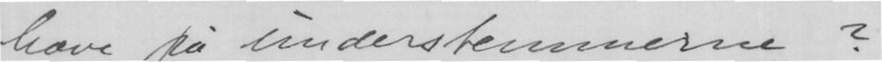have på understemmerne?
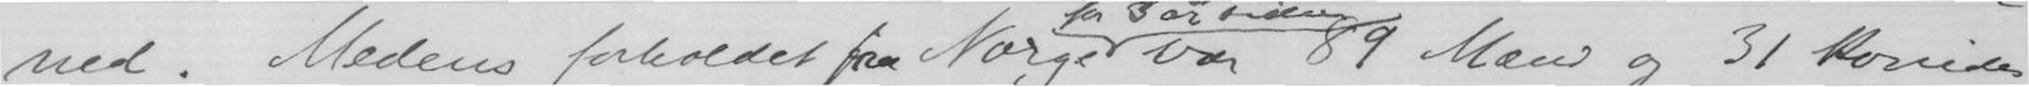ned. Medens forholdet fra Norge var 89 Mænd og 31 Kvinder
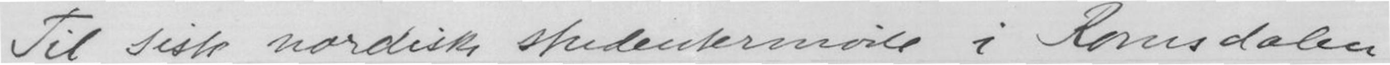Til siste nordiske studentermøde i Romsdalen
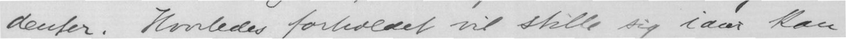denter. Hvorledes forholdet vil stille sig iaar kan
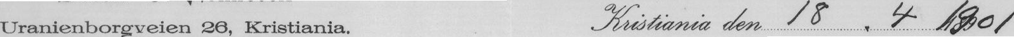Uranienborgveien 26, Kristiania Kristiania den 18.4 1901
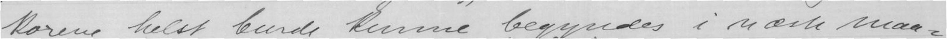korene helst burde kunne begyndes i næste maa-
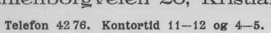Telefon 4276. Kontortid 11-12 og 4-5.
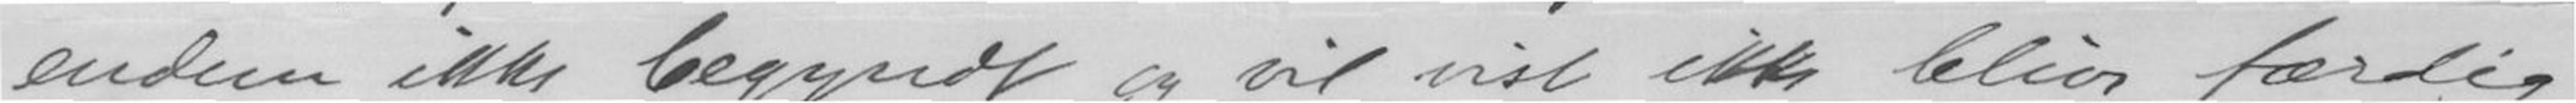endnu ikke begyndt og vil vist ikke blive færdig
